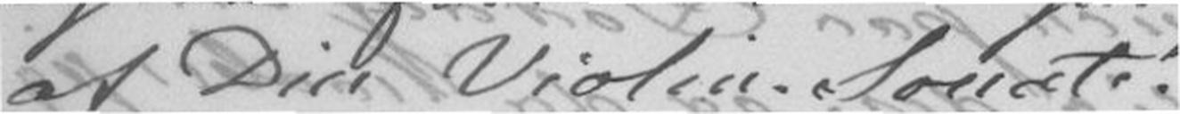af Din Violin Sonate.
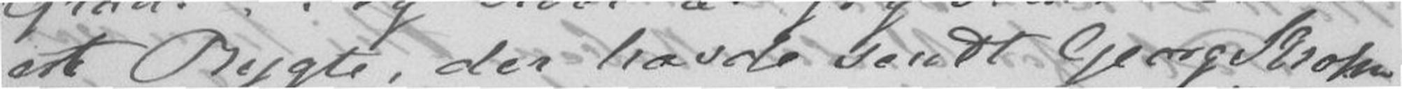et Rygte, der havde sendt Georg Krohn
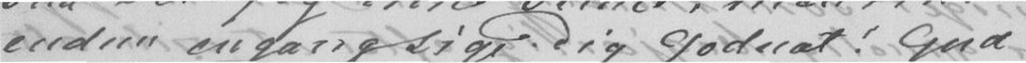endnu engang sige Dig Godnat! Gud
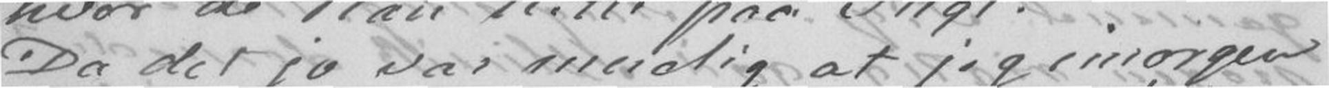Da det jo var muelig at jeg imorgen
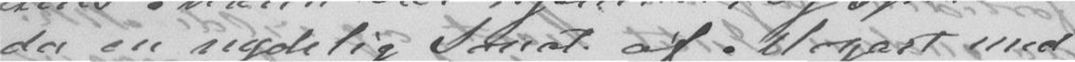der en nydelig Sonat af Mozart med
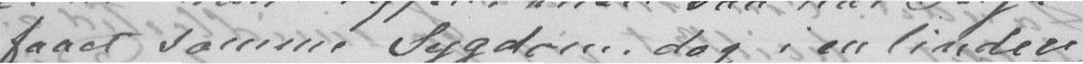faaet samme Sygdom, dog i en lindere
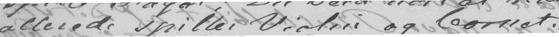allerede spiller Violin og Cornet.
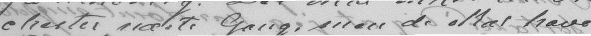chester næste Gang, men de skal have
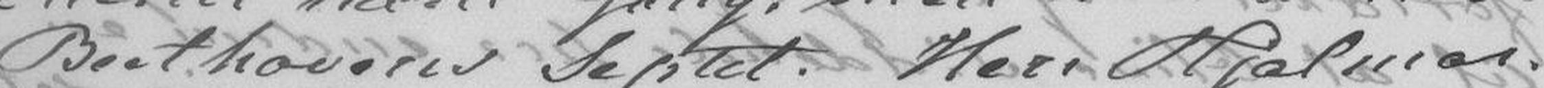Beethovens Septet. Herr Hjalmar
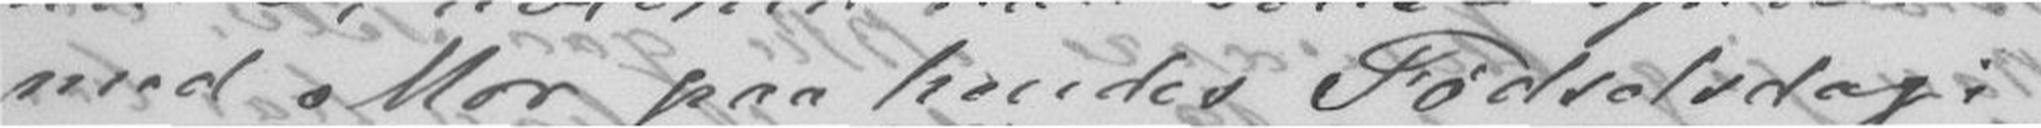med Mor paa hendes Fødselsdag;
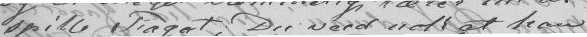spille Fagot; Du veed nok at han
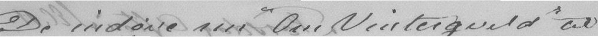De indøve nu "Om Vinterqveld! til
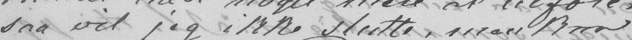saa vil jeg ikke slutte, men kun
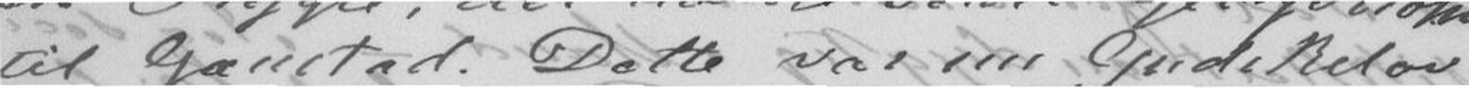til Gaustad. Dette var nu Gudskelov
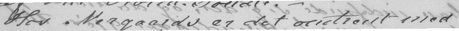Hos Nergaards er det omtrent med
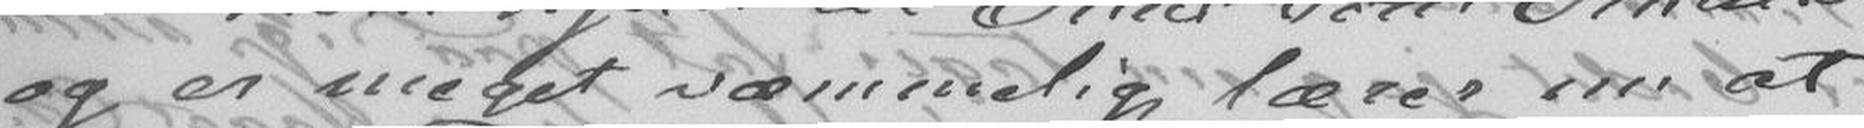og er meget væmmelig, lærer nu at
